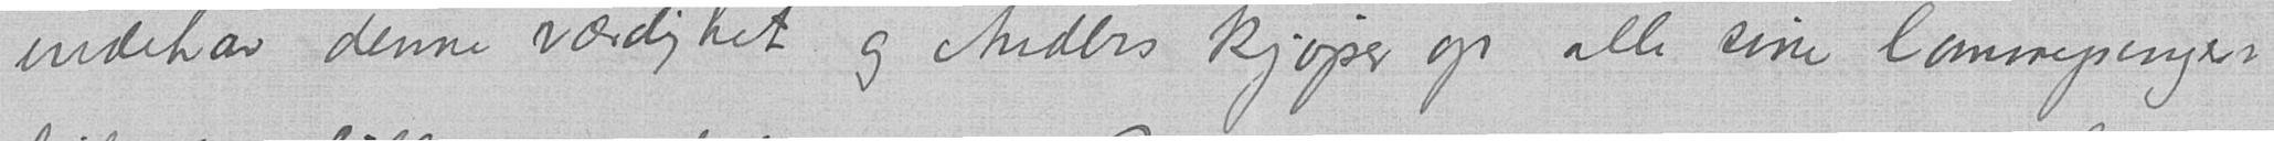indehar denne værdighet og Anders kjøper op alle sine lommepenger
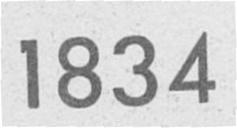1834
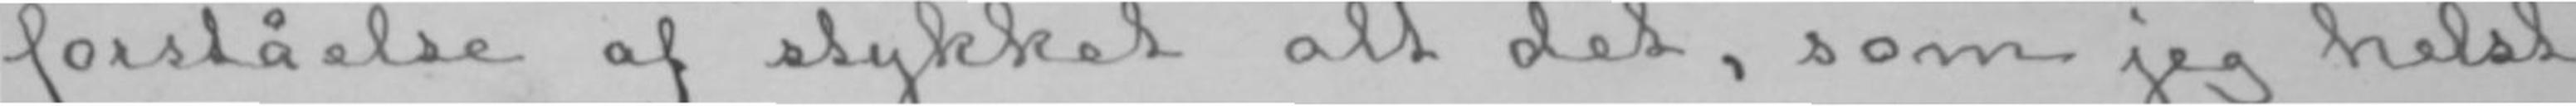forståelse af stykket alt det, som jeg helst
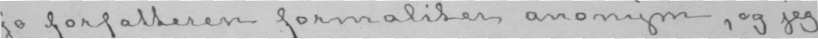jo forfatteren formaliter anonym, og jeg
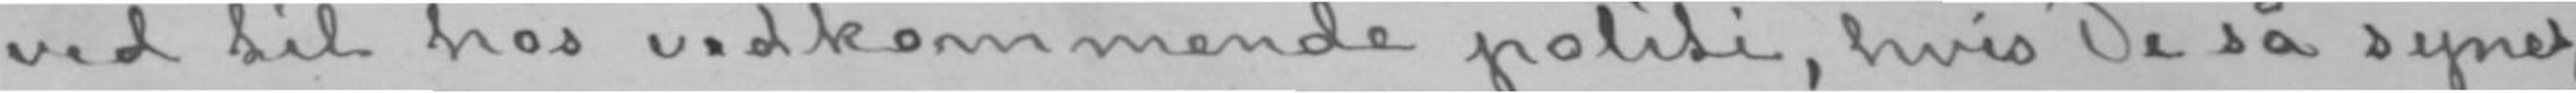ved til hos vedkommende politi, hvis De så synes,
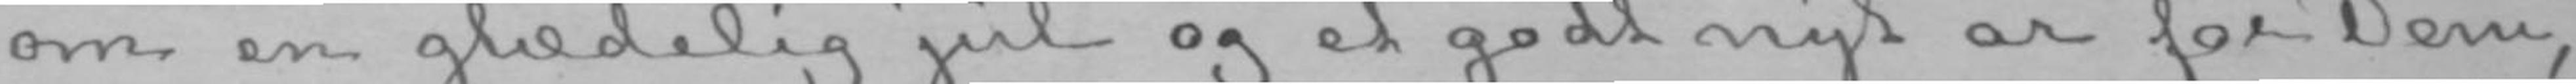om en glædelig jul og et godt nyt år for Dem,
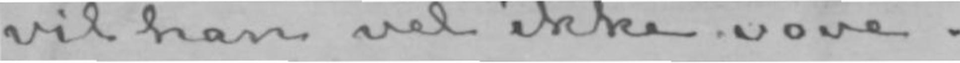vil han vel ikke vove.
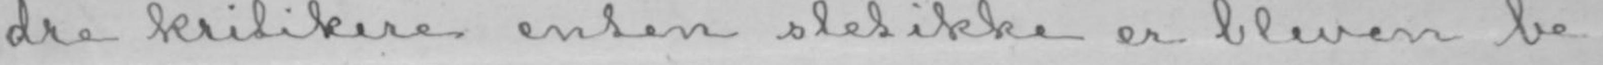dre kritikere enten sletikke er bleven be-
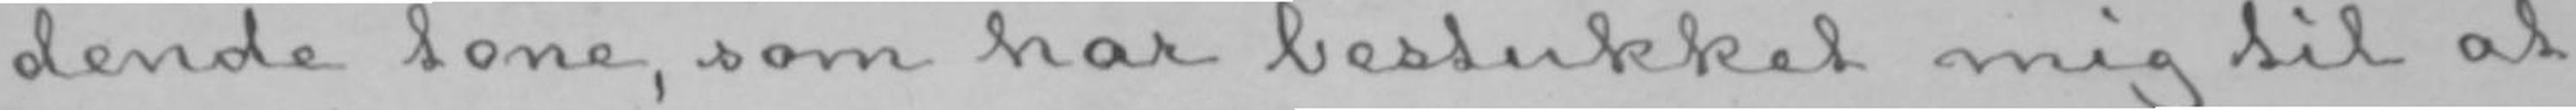dende tone, som har bestukket mig til at
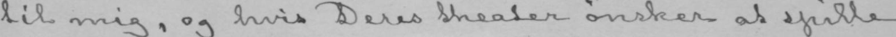til mig, og hvis Deres theater ønsker at spille
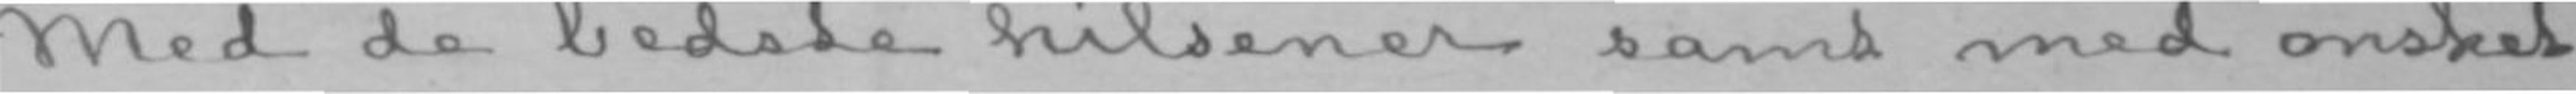Med de bedste hilsener samt med ønsket
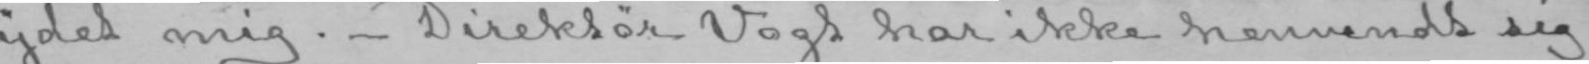ydet mig.- Direktør Vogt har ikke henvendt sig
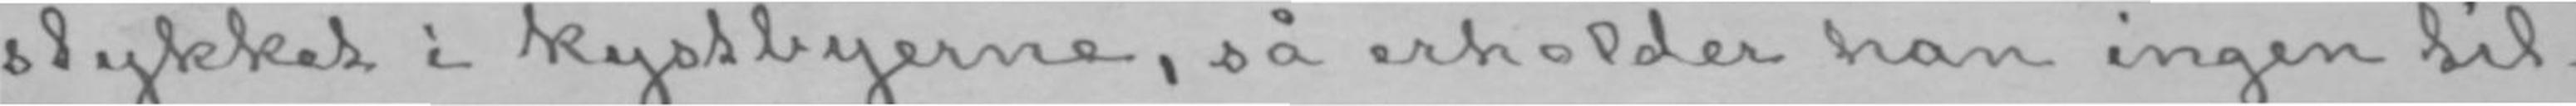stykket i kystbyerne, så erholder han ingen til-
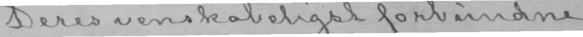Deres venskabeligst forbundne
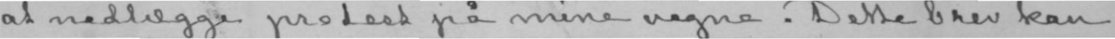at nedlægge protest på mine vegne. Dette brev kan
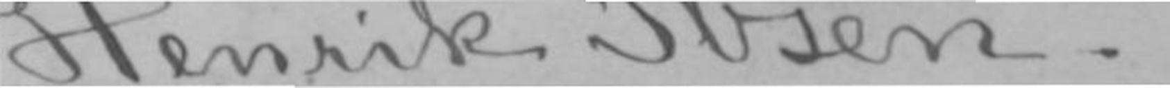Henrik Ibsen.
